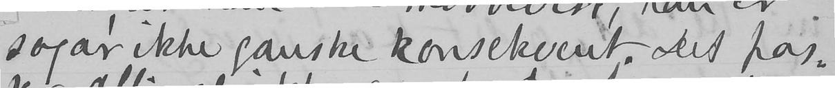sogar ikke ganske konsekvent. Det pas-
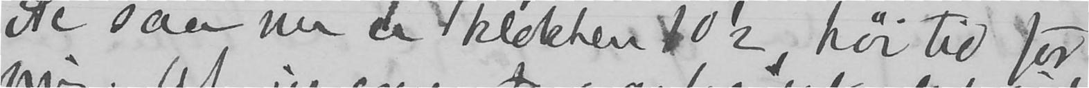Se saa nu er klokken 10 2, høi tid for
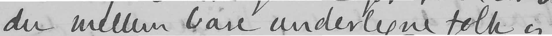der mellem bare underlegne folk og
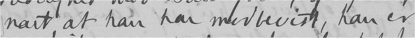nært, at han har modbevist, han er
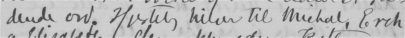dende ord. Hjertelig hilsen til Michael, Erik
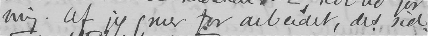mig at jeg gruer for arbeidet, det sid-
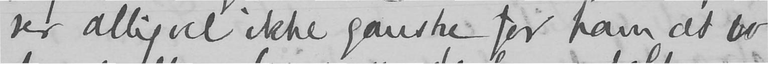ser alligevel ikke ganske for ham at bo
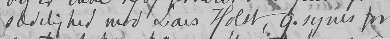sædelighed mod Lars Holst, G. synes for
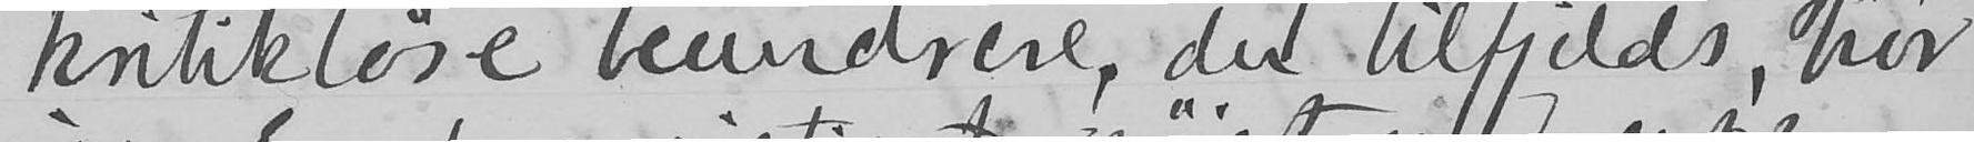kritikløse beundrere, der tilfjelds, tror
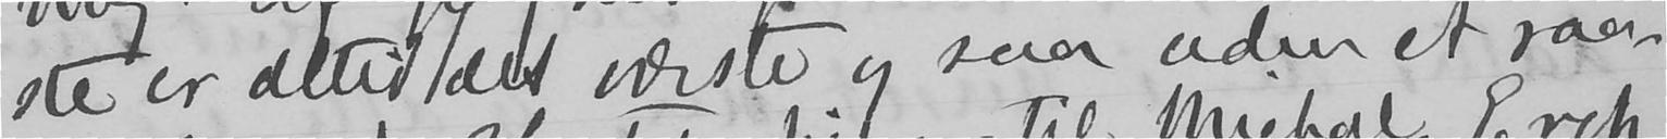ste er altid det værste og saa uden et raa-
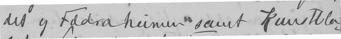det og Fædraheimen samt Kunstbla-
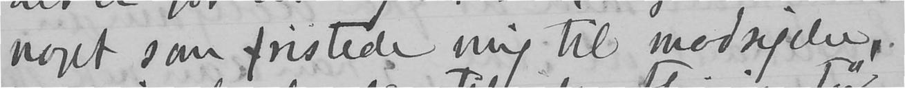noget som fristede mig til modsigelse,
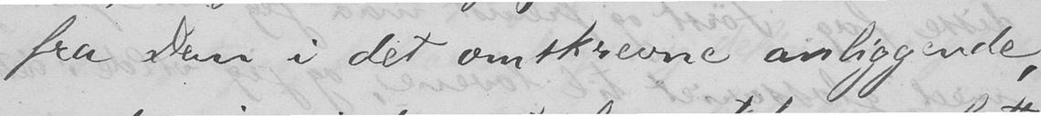fra Dem i det omskrevne anliggende,
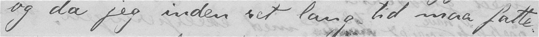og da jeg inden ret lang tid maa fatte
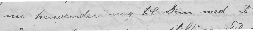nu henvender mig til Dem med et
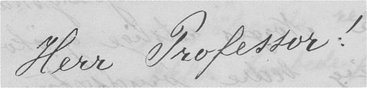Herr Professor!
